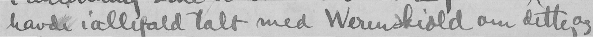havde iallefald talt med Werenskiold om dette, og
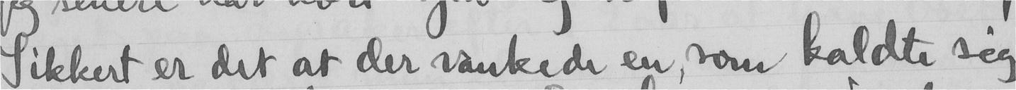Sikkert er det at der vankede en, som kaldte sig
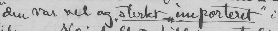den var vel og "sterkt  importeret" i
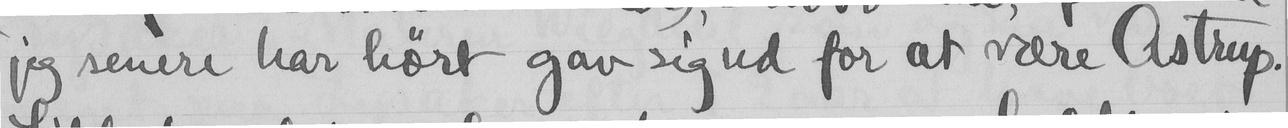jeg senere har hørt gav sig ud for at være Astrup.
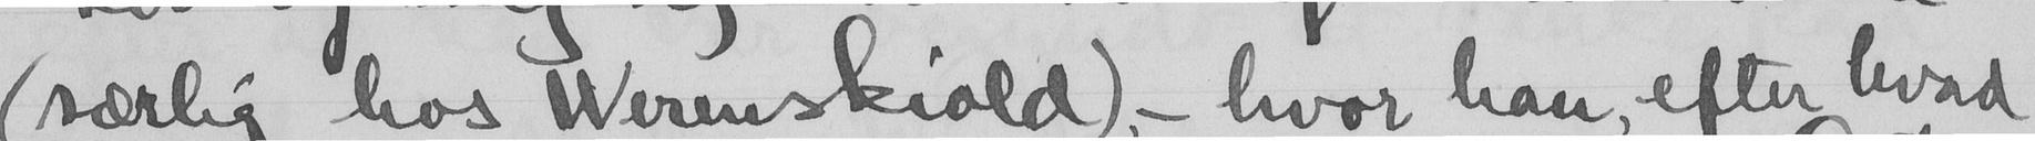(særlig hos Werenskiold), - hvor han, efter hvad
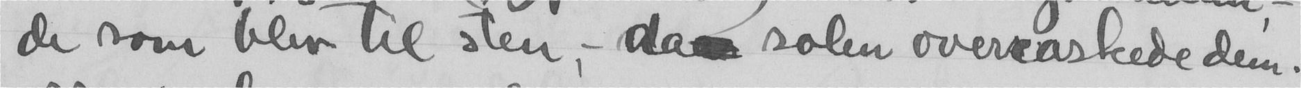de som blev til sten - da solen overraskede dem.
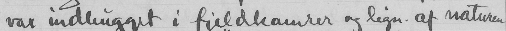var indhugget i fjeldkamrer og lign. af naturen
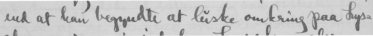end at han begyndte at luske omkring paa Lys-
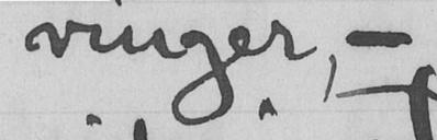vinger -
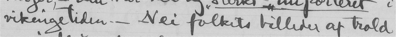vikingetiden. - Nei folkets billeder af trold
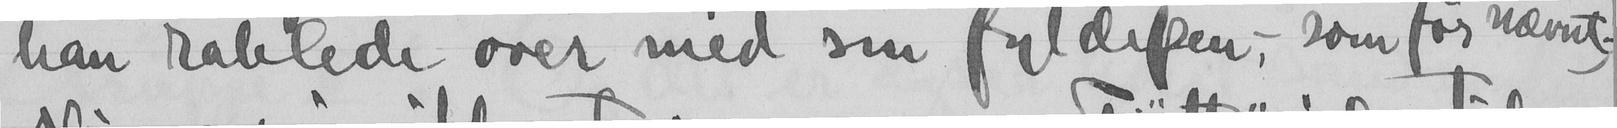han rablede over med sin fyldepen,- som før nævnt.)
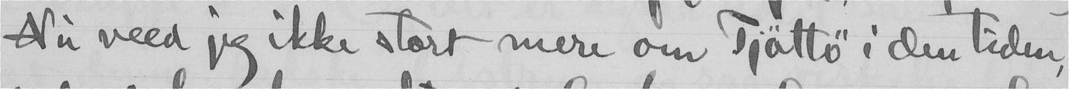Nu veed jeg ikke stort mere om Tjøttø i den tiden,
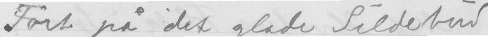Først på det glade Sildebud
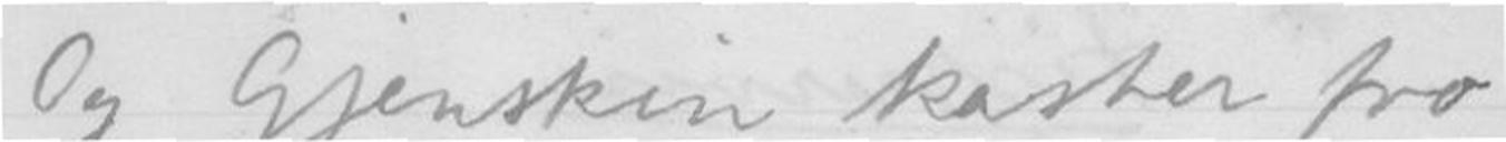Og gjenskin kaster fro
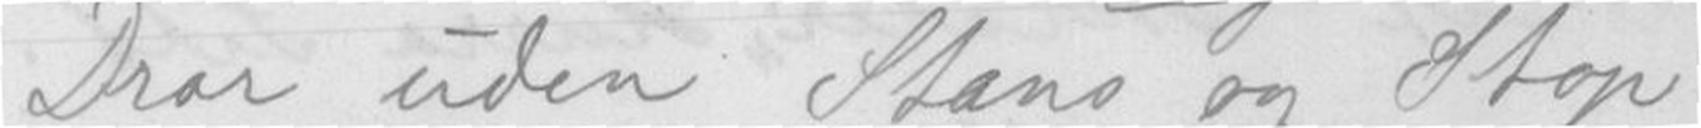Drar uden Stans og Stop
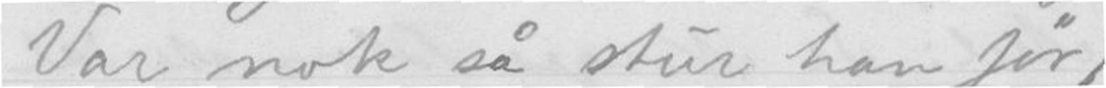Var nok så stur han før,
